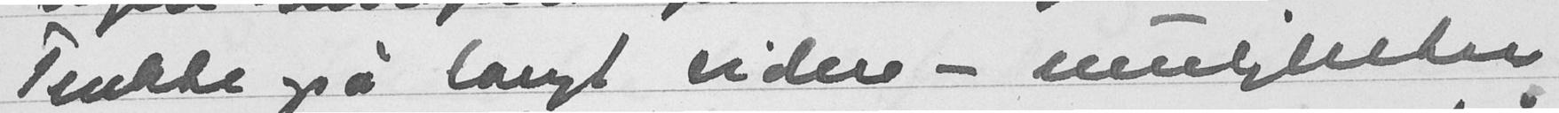Tenkte også langt videre - muligheter
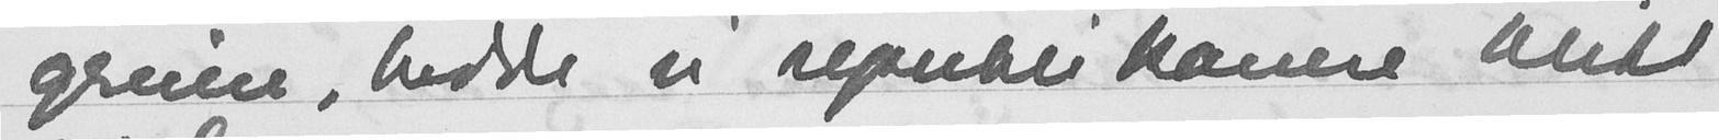greiere, hadde vi republikanere blitt
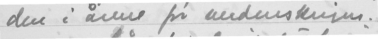den i årene før verdenskrigen.
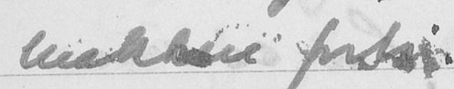makten forbi.
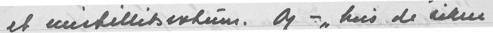et mistillitsvotum. Og - "hvis de sikrer
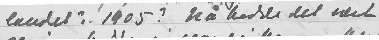landet i 1905? Nå hadde det vært
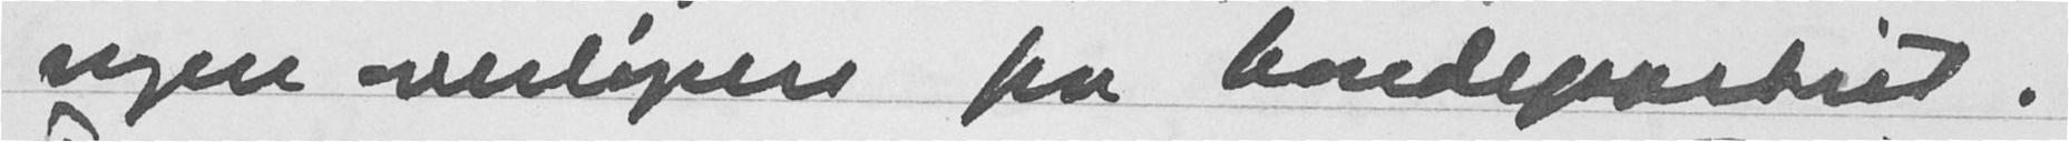nogen muligheter fra hovedpartiet.
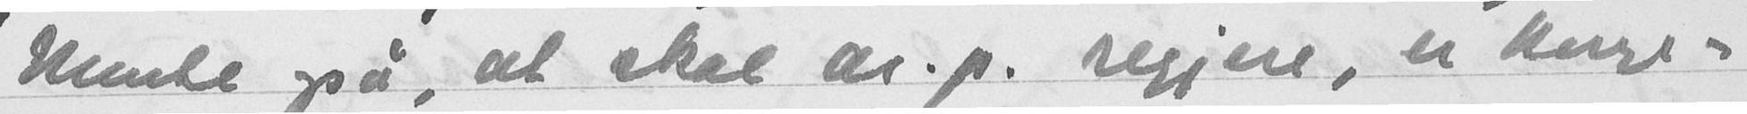Mente også, at skal ar. p. regjere, er konge-
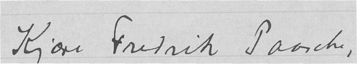Kjære Fredrik Paasche,
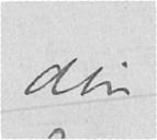din
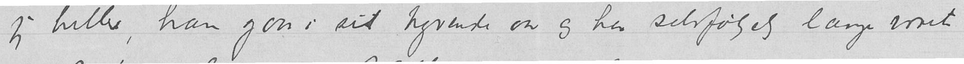jeg heller, han gaar i sit tyvende aar og har selvfølgelig længe været
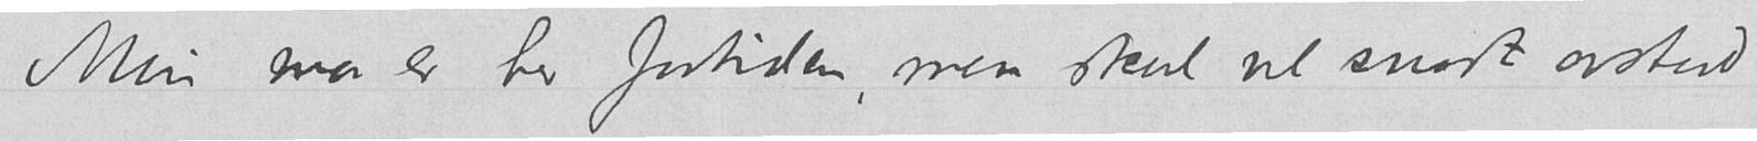Min mor er her fortiden, men skal vel snart avsted
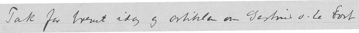Tak for brevet idag og artiklen om Gertrud v. le Fort
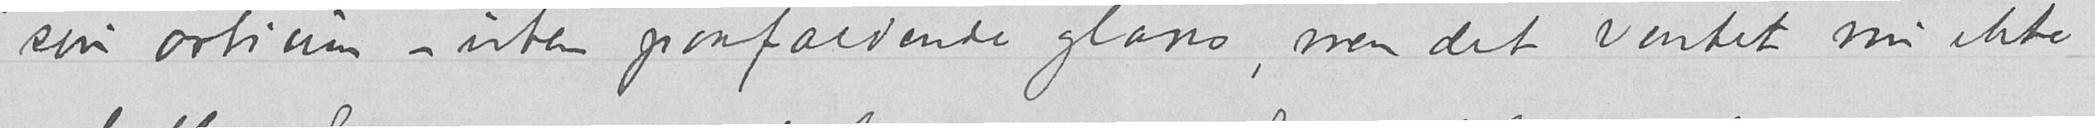sin artium – uten paafaldende glans, men det ventet nu ikke
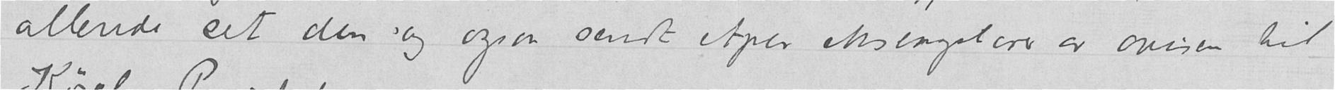allerede set den og ogsaa sendt etpar eksemplarer av avisen til
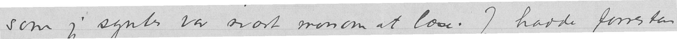som jeg syntes var svært morsom at læse. Jeg havde forresten
